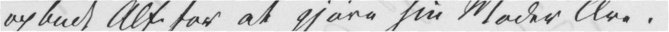opbudt Alt for at gjøre sin Moder Ære.
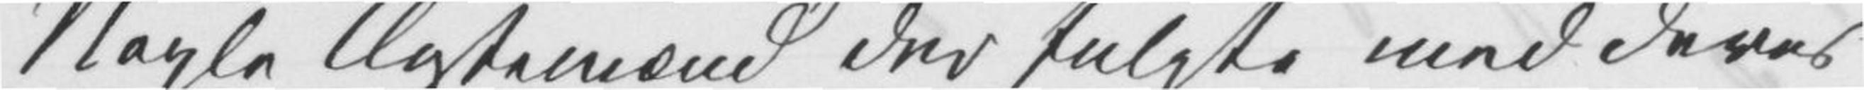Nogle Ægtemænd der fulgte med deres
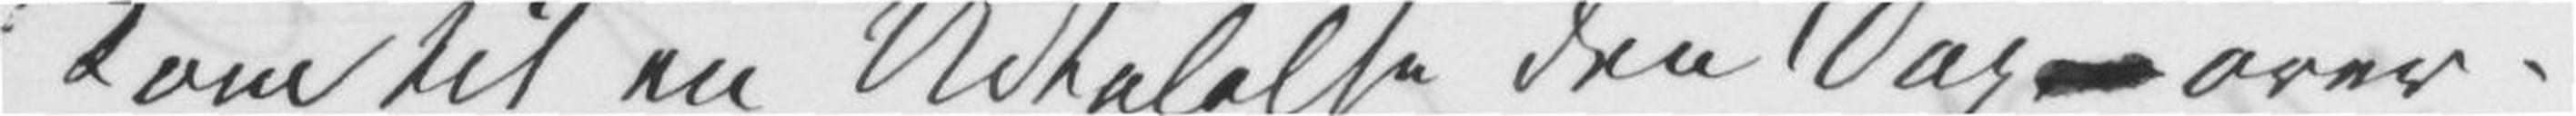kom til en Udtalelse den Dag – over¬
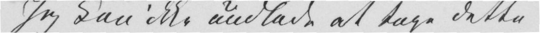Jeg kan ikke undlade at tage dette
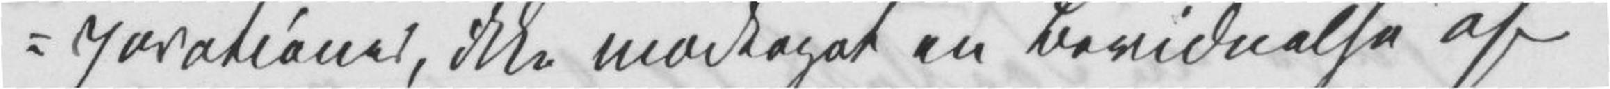porationer, ikke modtaget en Bevidnelse af
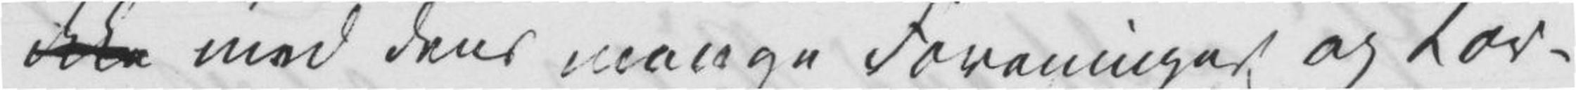ikke med dens mange Foreninger og Kor¬
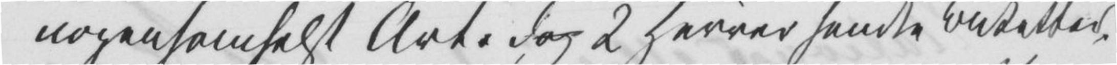nogensomhelst Art, dog 2 Herrer sendte Buketter.
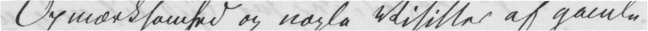Opmærksomhed og nogle Visitter af gamle
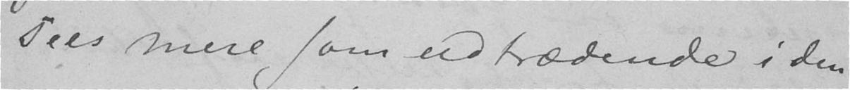sees mere som udtrædende i den
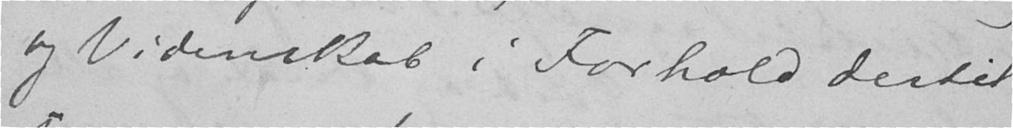og Videnskab i Forhold dertil
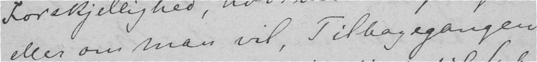eller, om man vil, Tilbagegangen
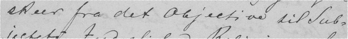skeer fra det Objective til Sub-
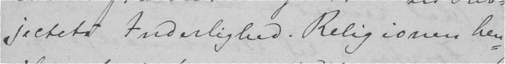jectets Inderlighed. Religionen hen-
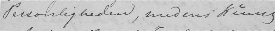Personligheden, medens Kunst
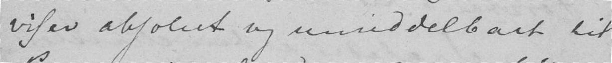viser absolut og umiddelbart til
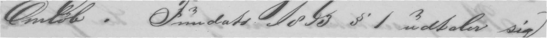Omløb. i Fundats. 1813 § 1 udtaler sig
